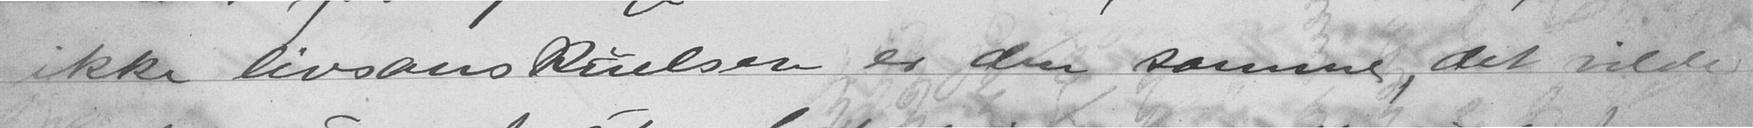ikke livsanskuelser er dem samme, det vilde
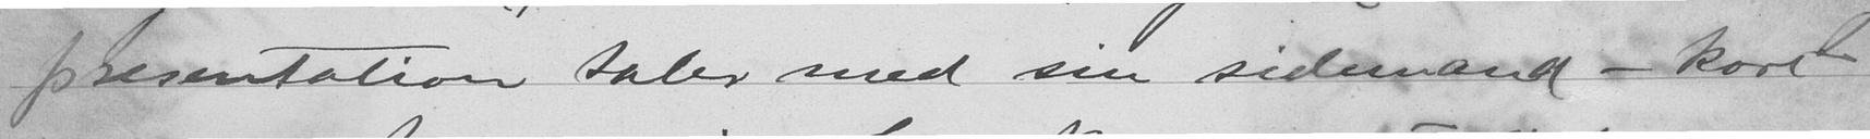presentation taler med sin sidemand - kort
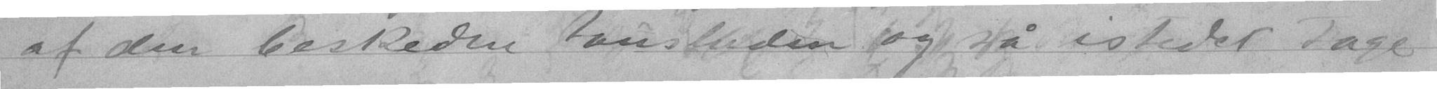af den beskeden tausheden og så istedet tage
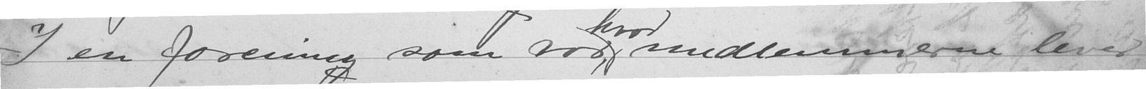I en forening som vår, hvor medlemmerne lever
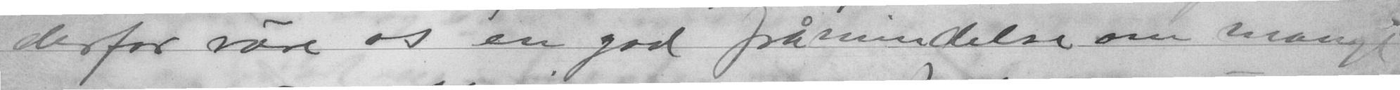derfor være os en god påmindelse om mangt
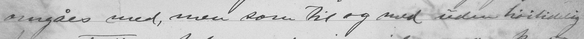omgåes med, men som til og med uden høitidelig
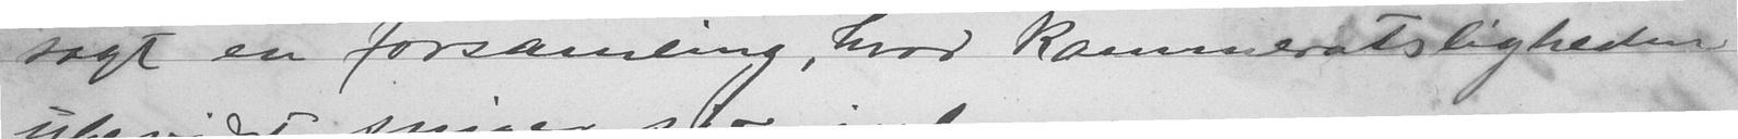sagt en forsamling, hvor kammeratsligheden
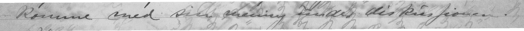komme med sin mening inden diskutionen.
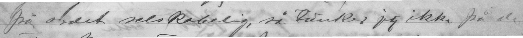på ordet selskabelig, så tenker jeg ikke på de
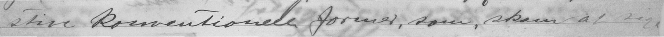store konventionelle former, som, skam at sige,
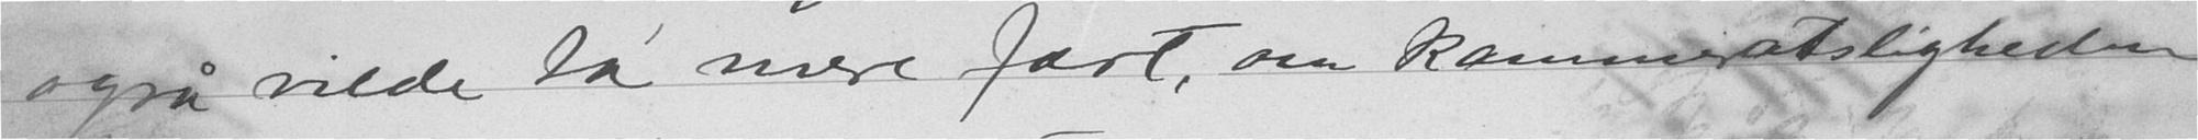også vilde ta mere fart, om kammeratsligheden
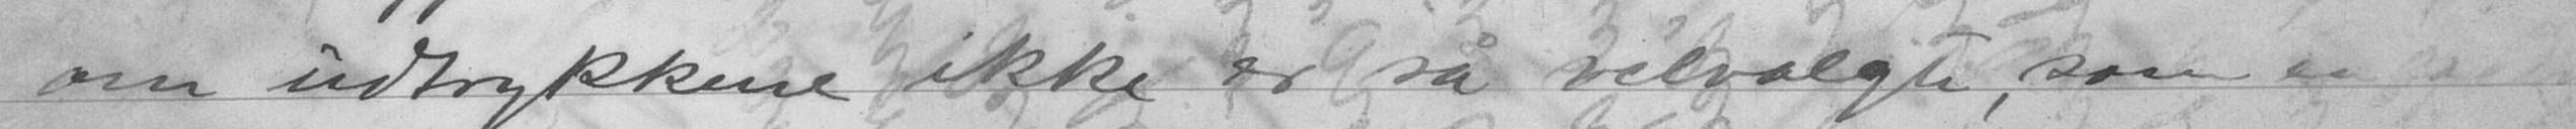om udtrykkene ikke er så velvalgte, som
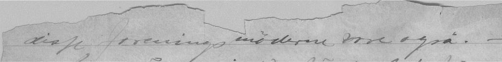disse foreningsmødene vore også. -
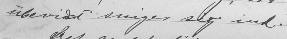ubevidst smiger sig ind.
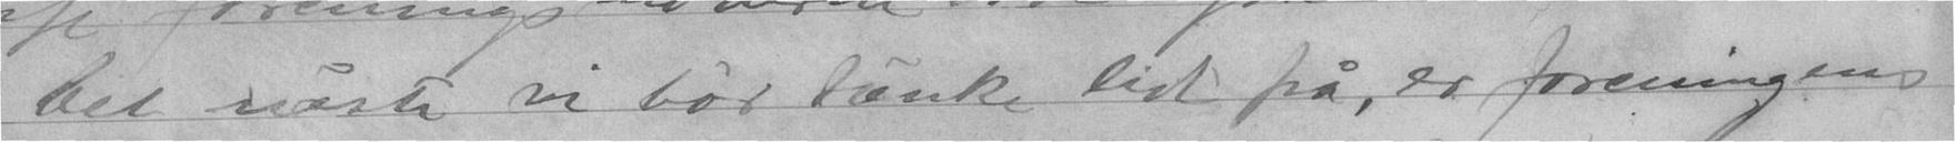let måske vi bør tenke lidt på, er foreningens
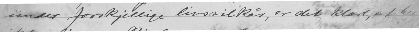under forskjellige livsvilkår, er det klart, at her
